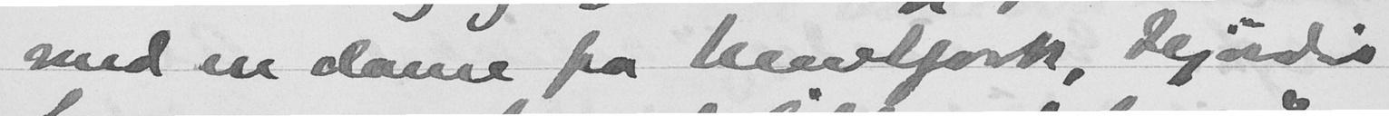med en dame fra Blevfjord, Hjørdis
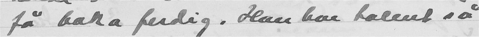få boka ferdig. Han har talent så
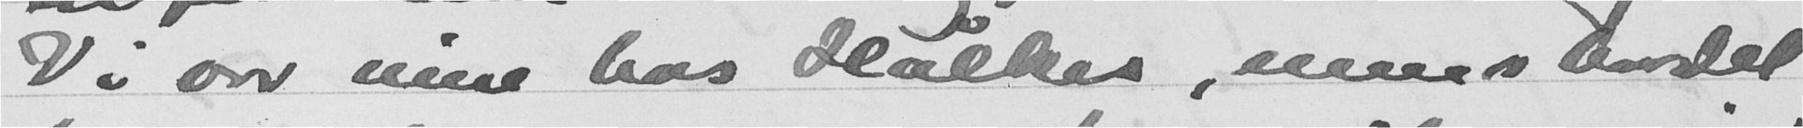Vi var inne hos Holkes, mens bordet
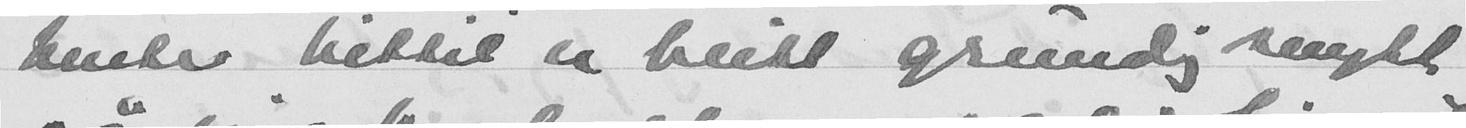benters hittil er blitt grundig snytt
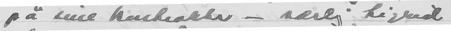på sine kontrakter - særlig Sigrid
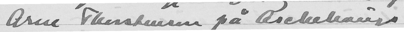Arne Thorstenson på Aschehaugs
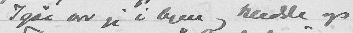Igår var jeg i byen og kledde op
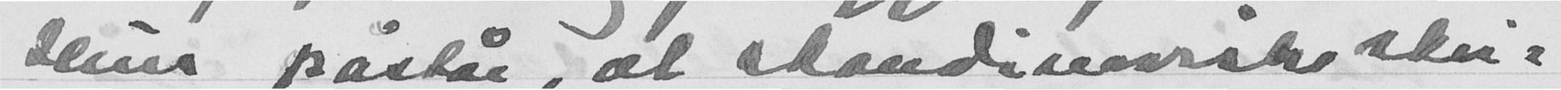Hun påstår, at skandinaviske skri-
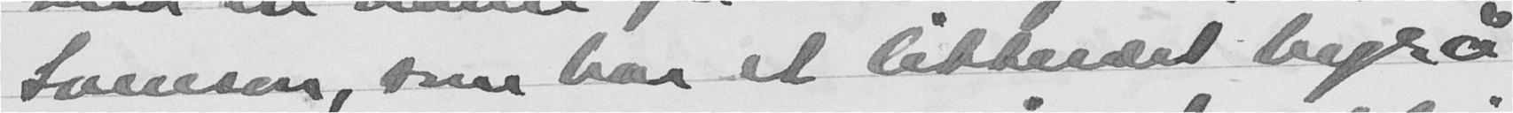Svenson, som har et litterært byrå
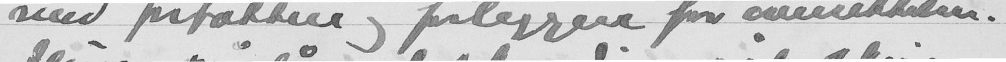med forfattere og forleggere for annettelser.
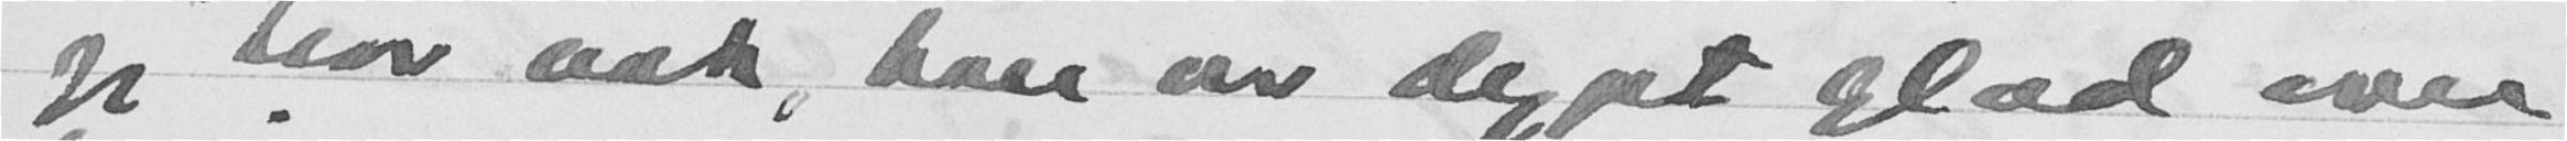jeg tror nok, han var dypt glad over
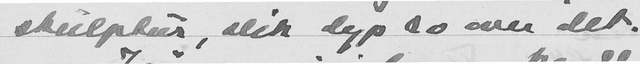skulptur, slik dyp ro over det.
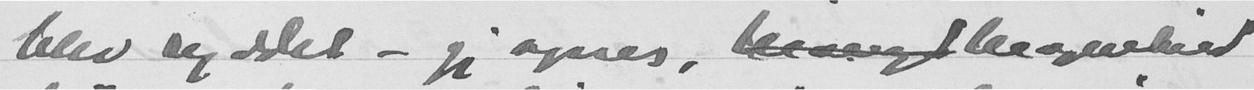blev ryddet - jeg synes,  lagenhild
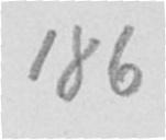186
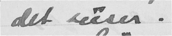det suser.
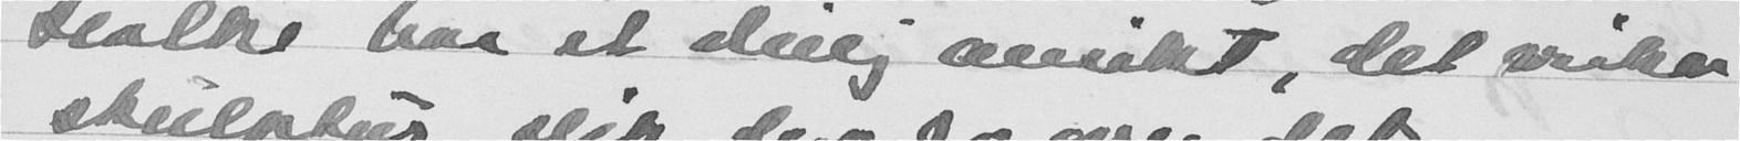Holke har et deilig ansikt, det virker
